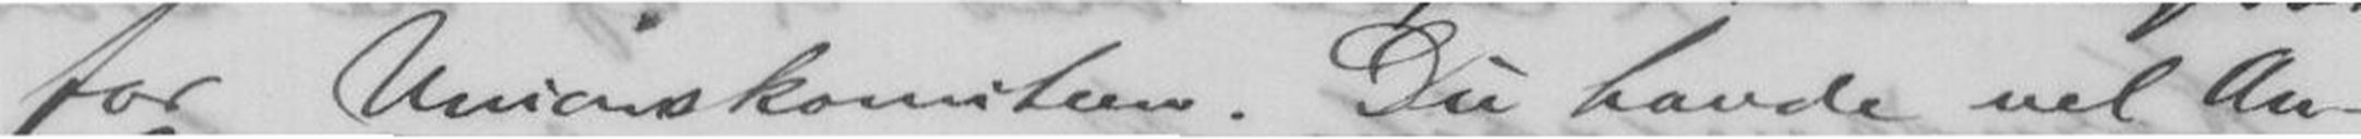for Unionskomiteen. Du havde vel An-
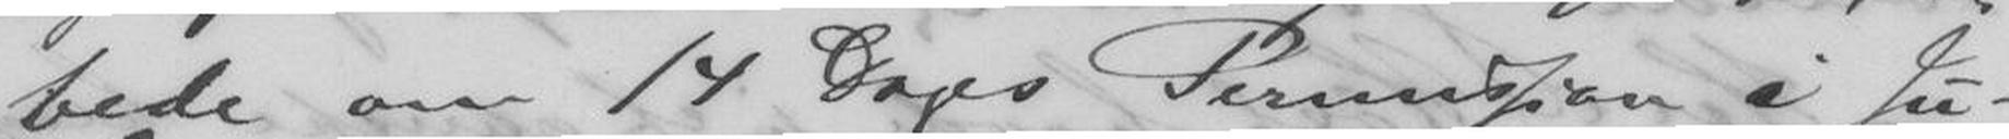bede om 14 Dages Permission i Ju-
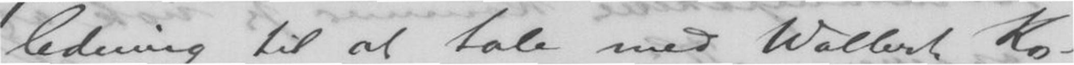ledning til at tale med Wollert Ko-
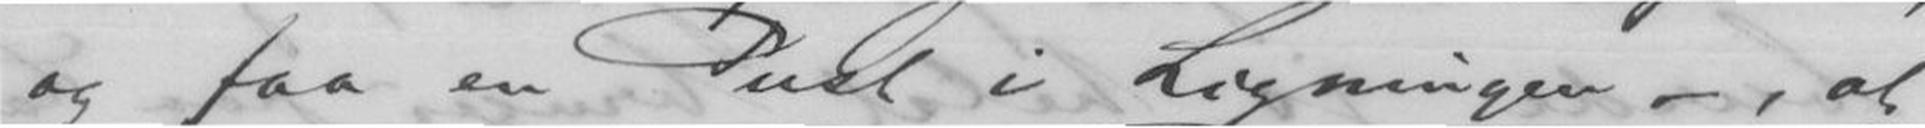og faa en Pust i Ligningen -, at
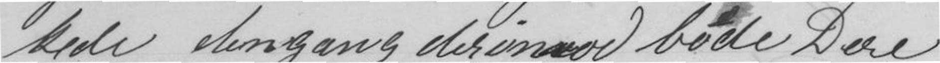kede dengang derimod bode Dere
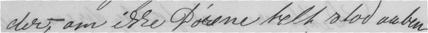der, - om ikke Dørene helt stod aaben
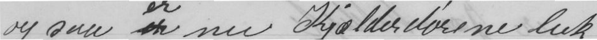og som er nu Kjælderdørene luk-
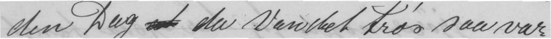den Dag da Vandet Frøs saa var
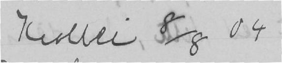Kvalbein 8/8 04
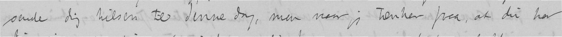sende dig hilsen til denne dag men naar jeg tænker paa, at du har
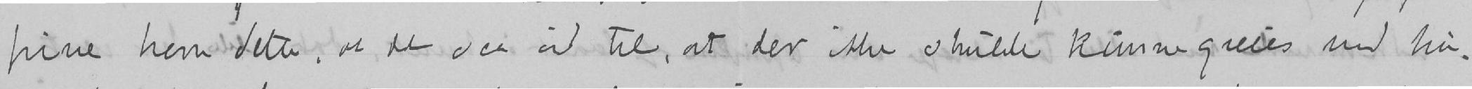pine kom dette, at det saa ud til, at der ikke skulle kunne greies med hu-
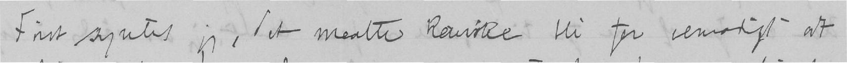Først syntes jeg, det maatte kanske bli for vemodigt at
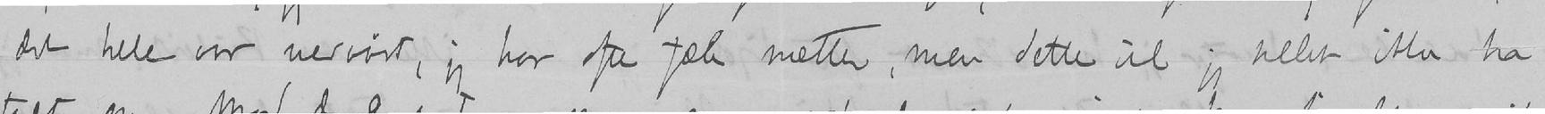det hele var nervøst, jeg har ofte fæle netter, men dette vil jeg heller ikke ha
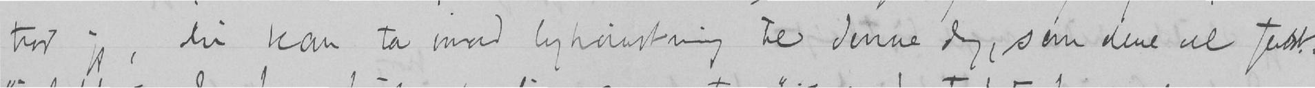tror jeg, du kan ta imod lykønskning til denne dag, som dere vel fæst-
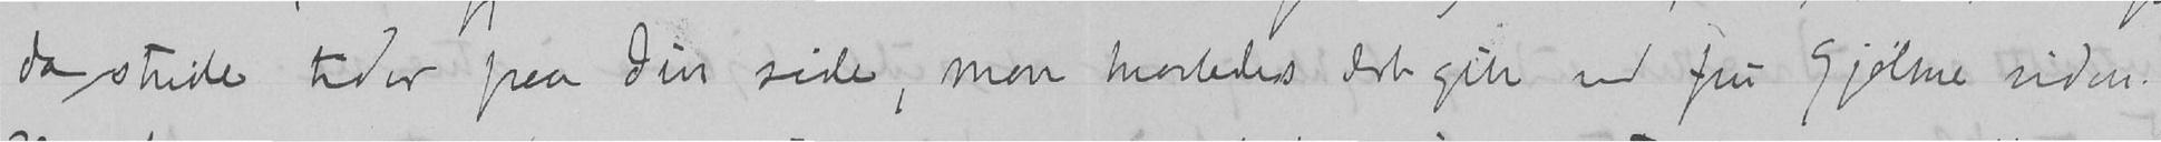da stride tider paa Din side, mon hvorledes det gik med fru Gjølme siden.
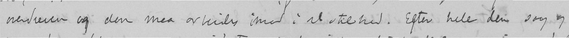overdreven og den maa arbeides imod i al stilhed. Efter hele den sorg og
